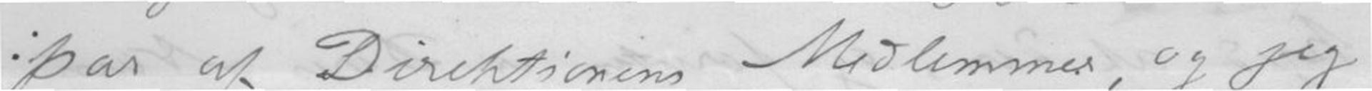par af Direktionens Medlemmer, og jeg
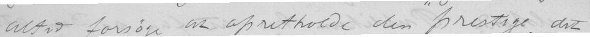altid forsøge at opretholde den "prestige", det
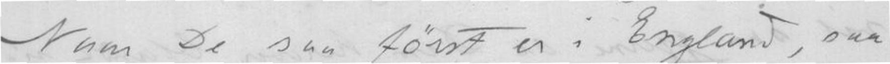Naar De saa først er i England, saa
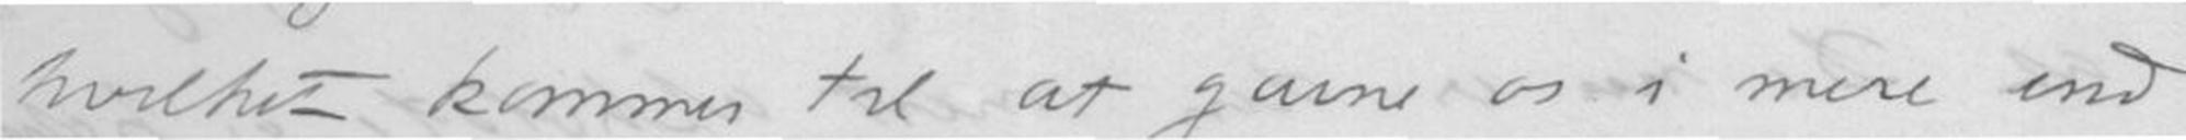hvilket kommer til at gavne os i mere end
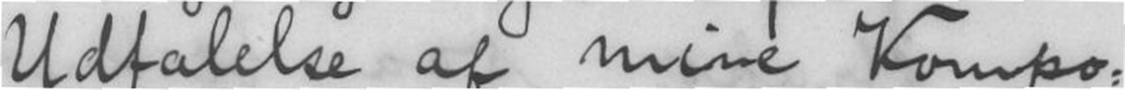Udtalelse af mine Kompo-
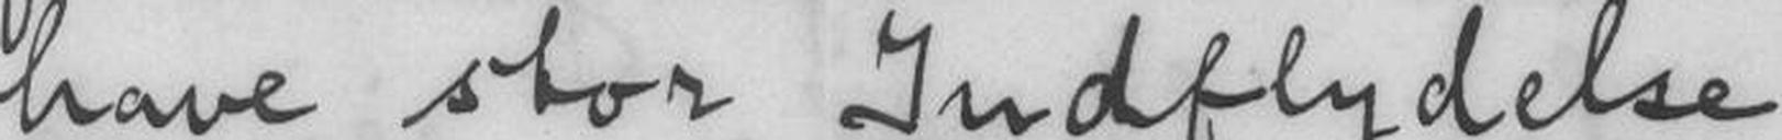have stor Indflydelse
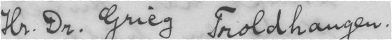Hr. Dr. Grieg Troldhaugen.
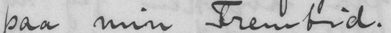paa min Fremtid.
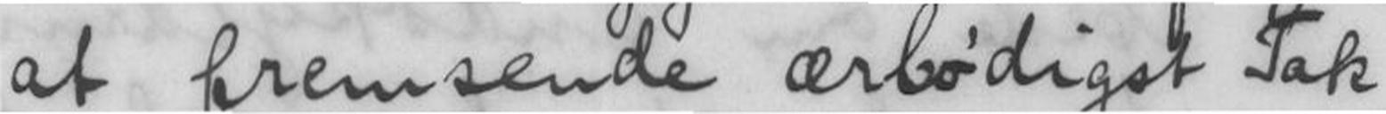at fremsende ærbødigst Tak
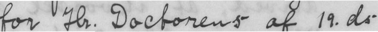for Hr. Doctorens af 19. ds.
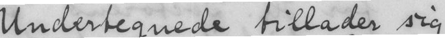Undertegnede tillader sig
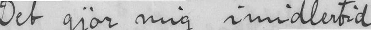Det gjør mig imidlertid
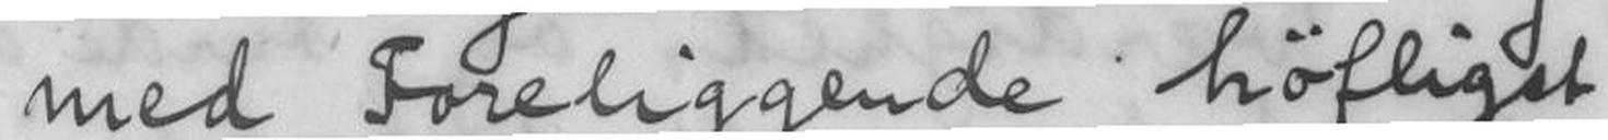med Foreliggende jøfligst
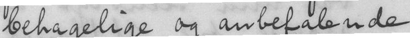behagelige og anbefalende
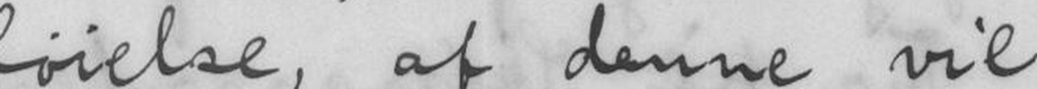førelse, af denne vil
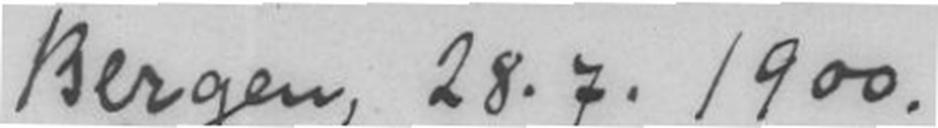Bergen, 28.7.1900.
